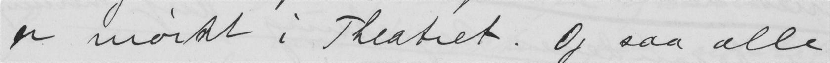er mørkt i Theatret. Og saa alle
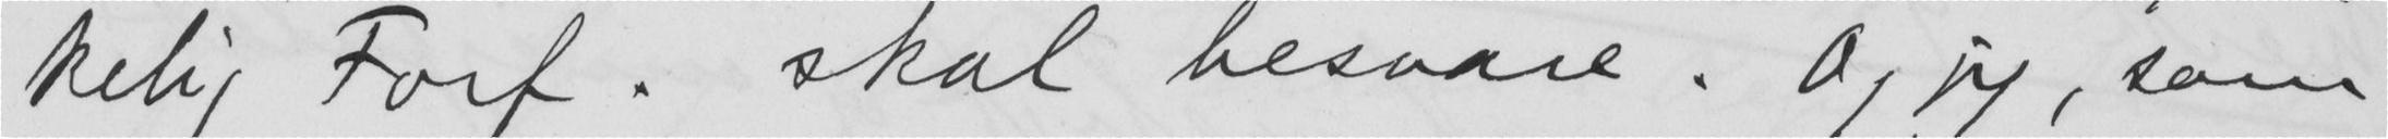kelig Forf. skal besvare. Og jeg, som
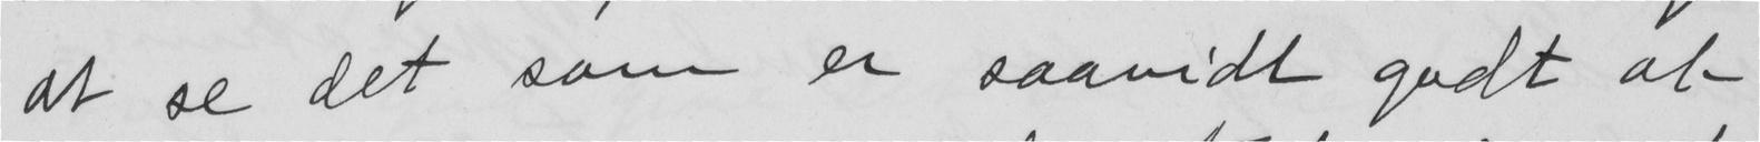at se det som er saavidt godt at
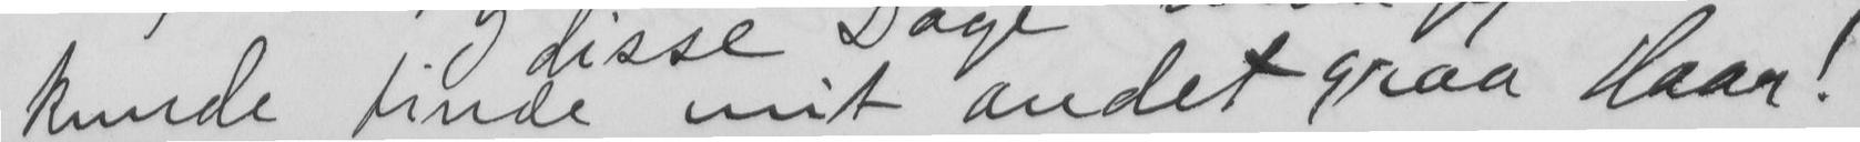kunde finde mit andet graa Haar!
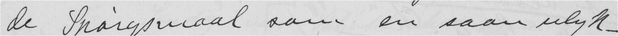de Spørgsmaal som en saan ulyk -
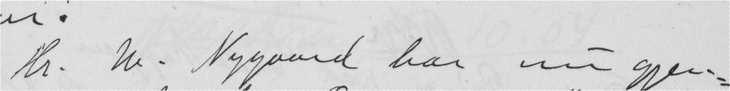Hr. W. Nygaard har nu gjen
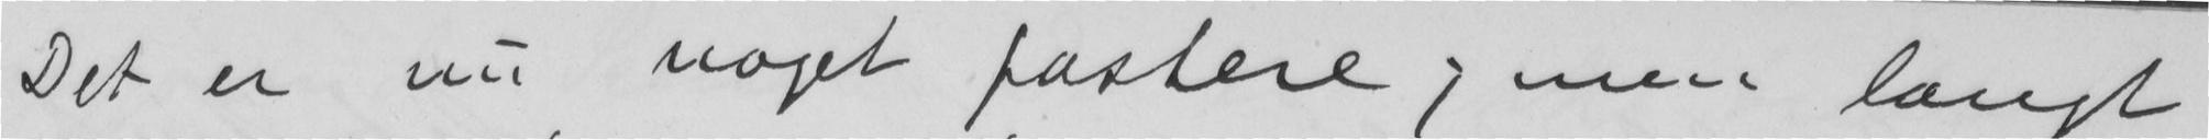Det er nu noget fastere; men langt
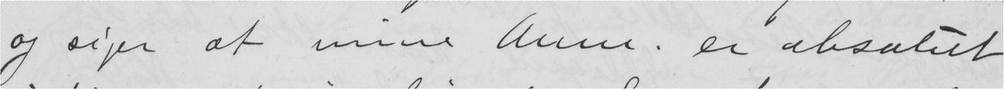og siger at mine Anm. er absolut
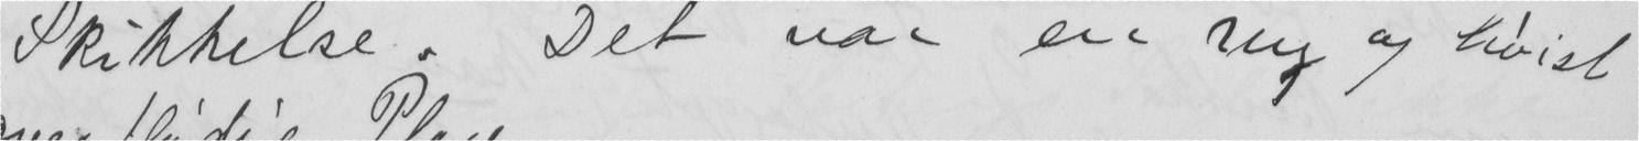Skikkelse. Det var en ny og høist
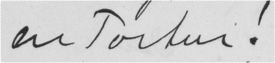en Tortur!
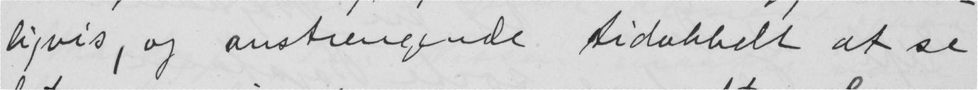ligvis, og anstrengende tidobbelt at se
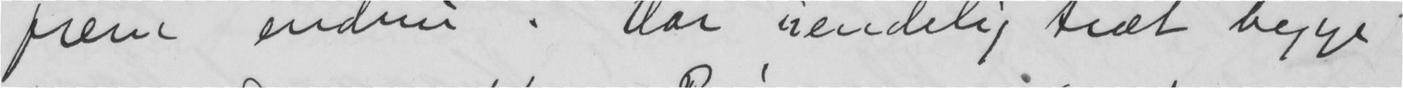frem endnu. Var uendelig træt begge
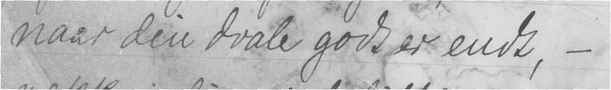naar den dvale godt er endt, -
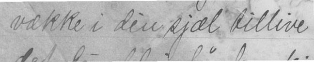vække i den sjæl tillive
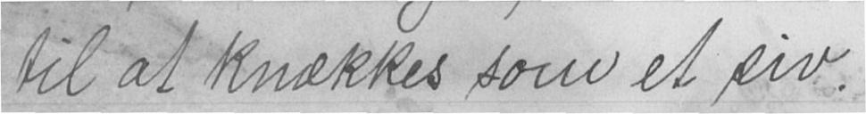til at knækkes som et siv.
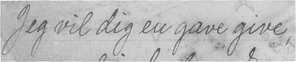Jeg vil dig en gave give,
